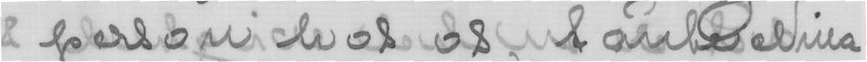person hos os, tante Nina
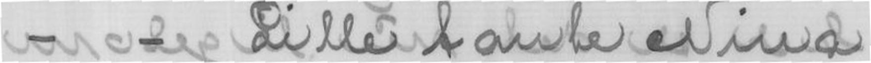- - Lille tante Nina
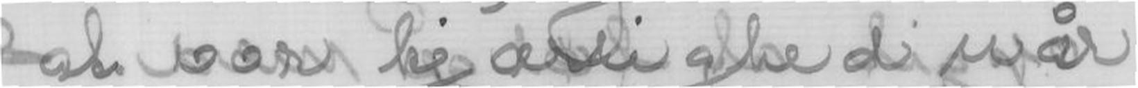at vor kjærlighed når
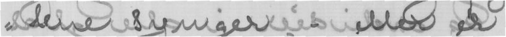lene synger, - eller er
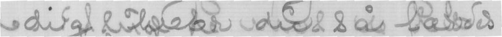dig, hvor du så færdes
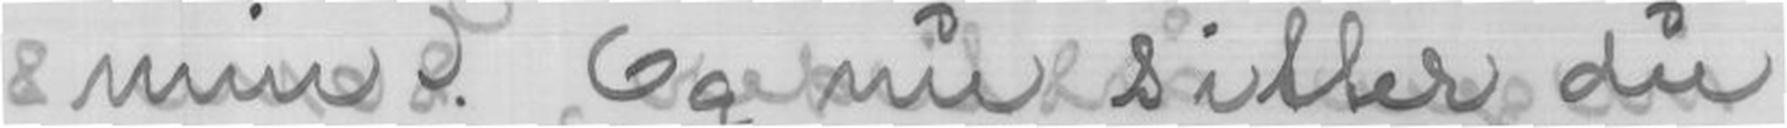min! Og nu sitter du
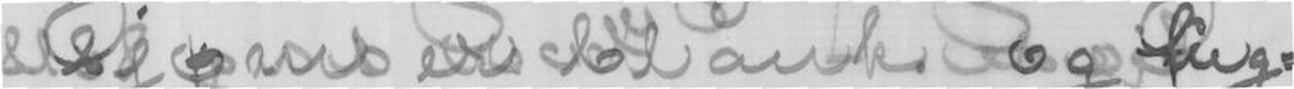sjøen er blank og fug-
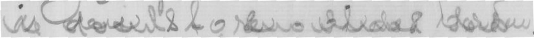i den store - store værden.
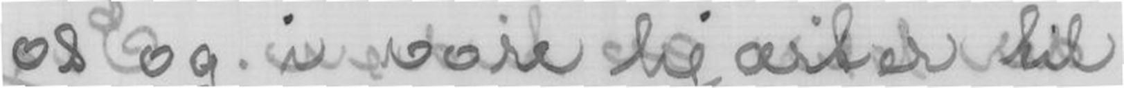os og i vore hjærter til
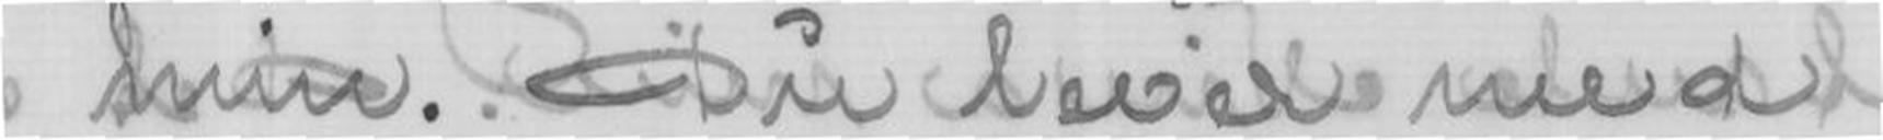min. Du lever med
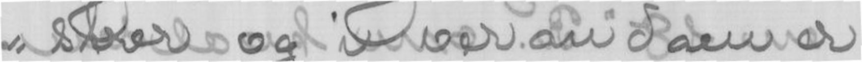strer og i verandaen er
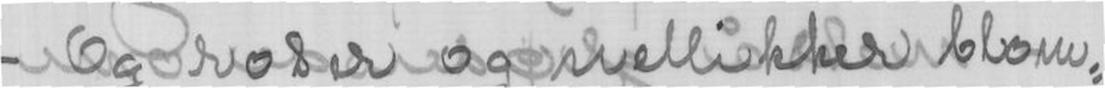- Og roser og nellikker blom-
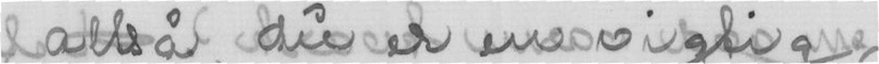altså, du er en vigtig
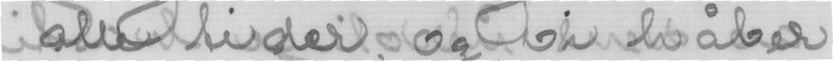alle tider, og vi håber
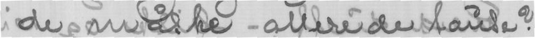de måske - allerede tause?
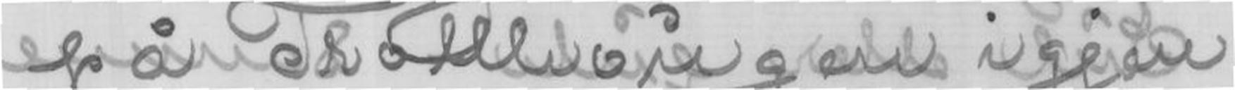på Trollhougen igjen,
